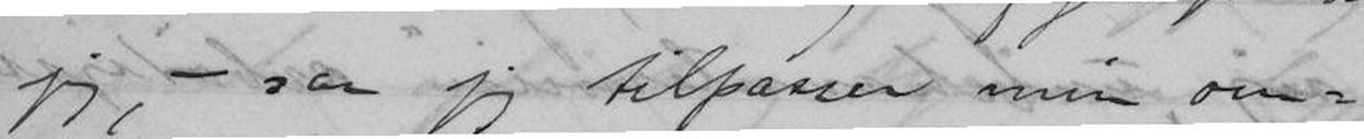jeg, - saa jeg tilpasser min om-
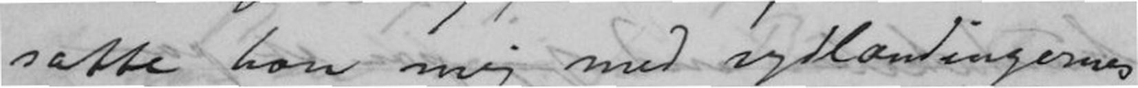satte han mig med sydlændingernes
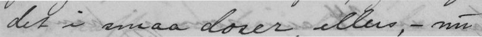det i smaa doser, ellers, - nu
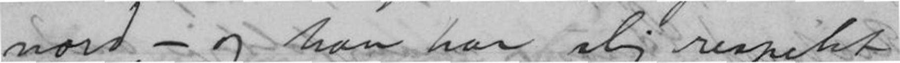nord, - og han har slig respekt
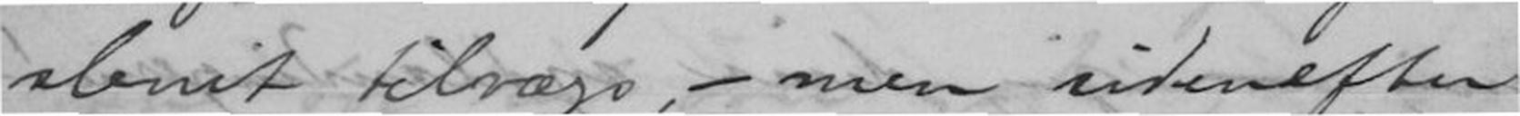slemt tilvægs, - men sidenefter
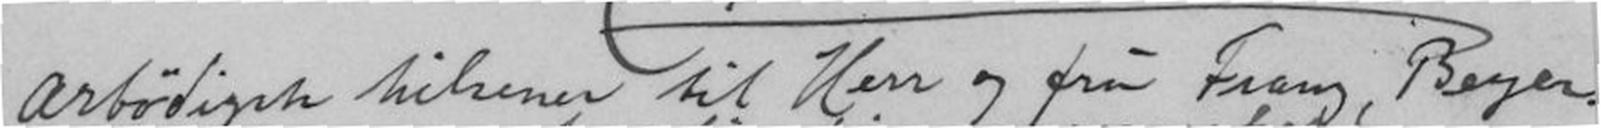Ærbødigste hilsener til Herr og fru Franz Beyer!
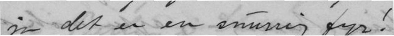ja det er en snurrig fyr! -
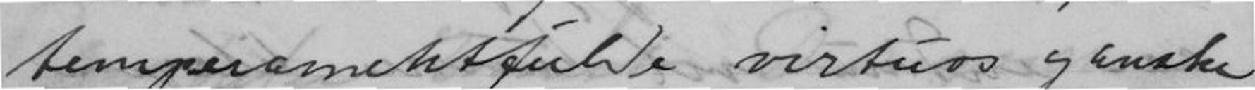temperamentfulde virtuos ganske
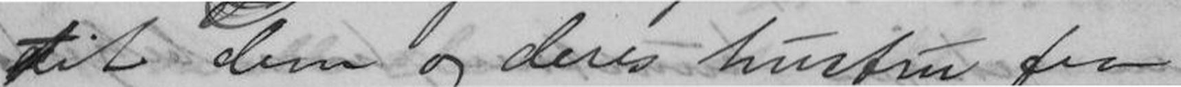til dem og deres hustru fra
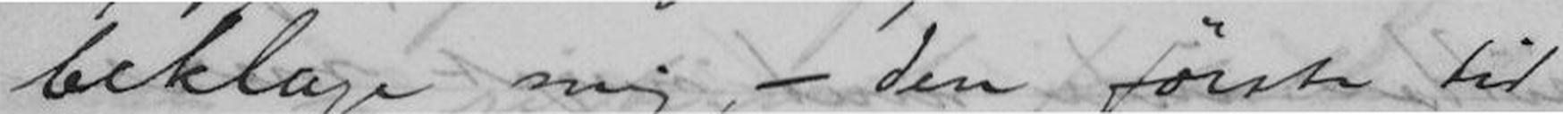beklage mig, - den første tid
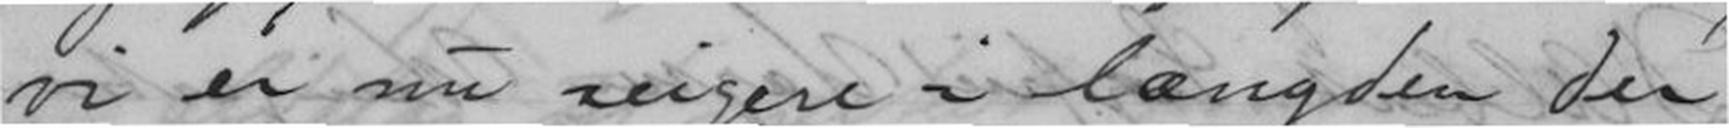vi er nu seigere i længden der
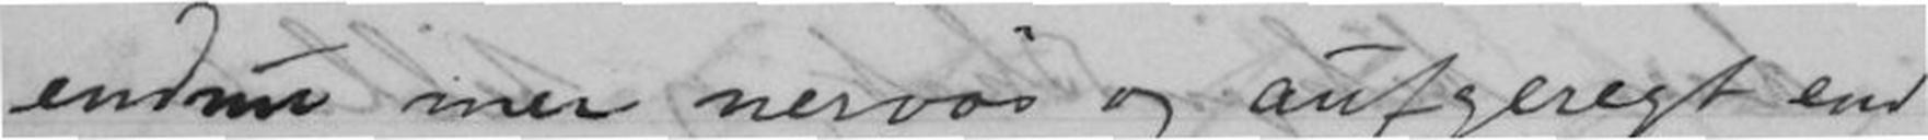endnu mer nervøs og aufgeregt end
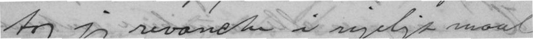tog jeg revanche i rigeligt maal,
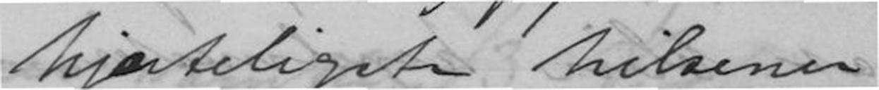hjærteligste hilsener
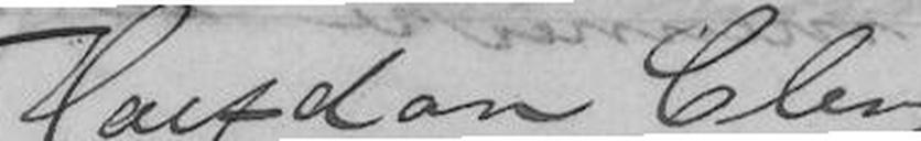Halfdan Cleve
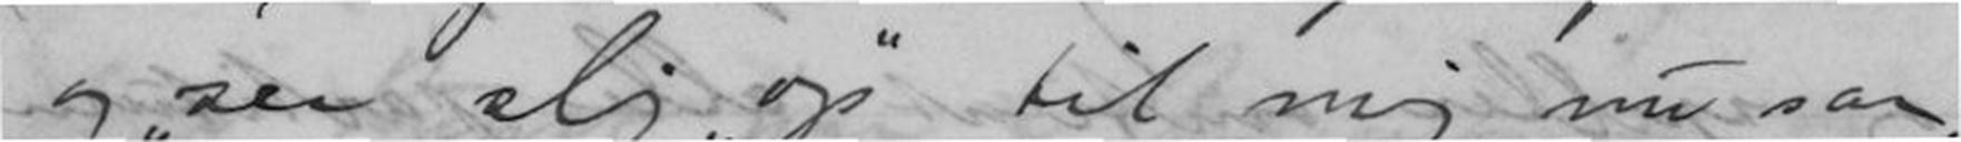og "ser slig "op" til mig nu saa,
